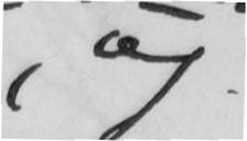, og
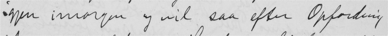igjen imorgen og vil saa efter Opfordring
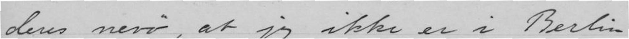deres nevø, at jeg ikke er i Berlin
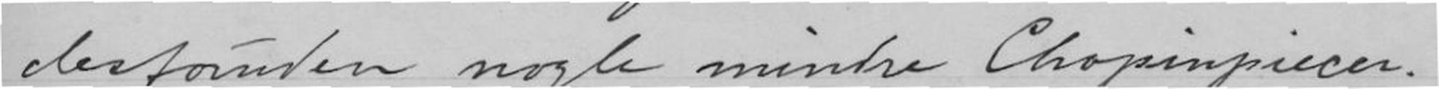desforuden nogle mindre Chopinpiecer.
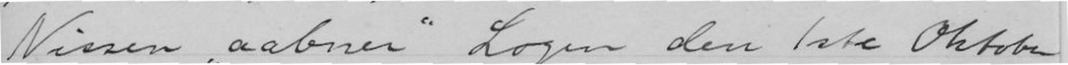Nissen aabner Logen den 1ste Oktober
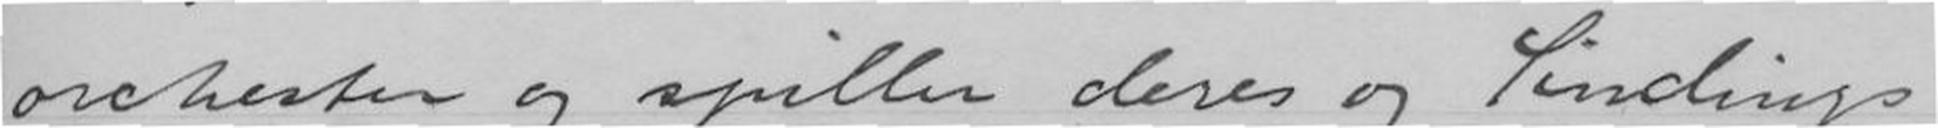orchester og spiller deres og Sindings
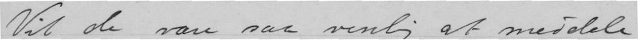Vil de være saa venlig at meddele
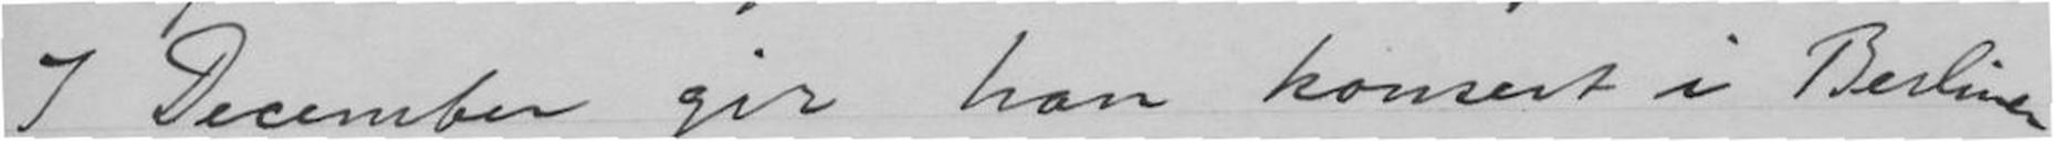7 Desember gir han konsert i Berliner
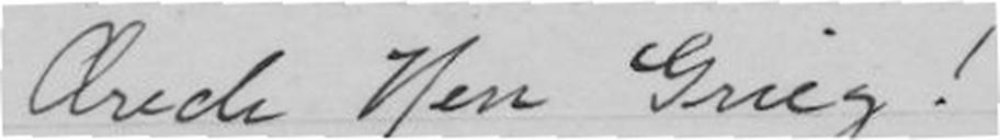Ærede Herr Grieg!
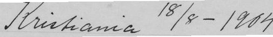Kristiania 18/8-1904.
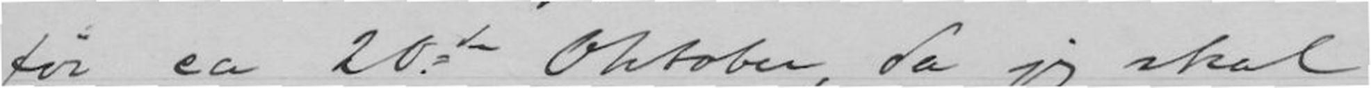før ca 20de Oktober, da jeg skal
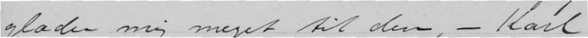glæder mig meget til den, - Karl
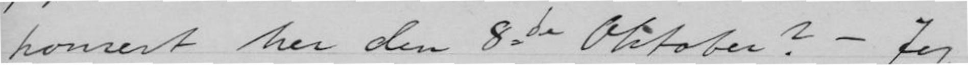konsert her den 8de Oktober? - Jeg
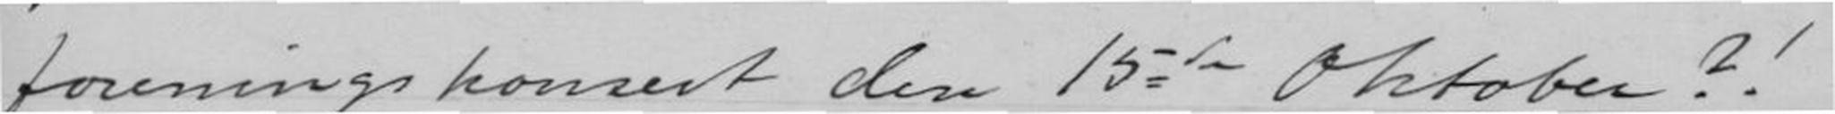foreningskonsert den 15de Oktober?!
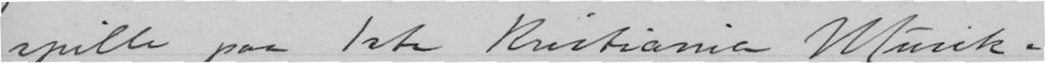spille paa 1ste Kristiania Musik-
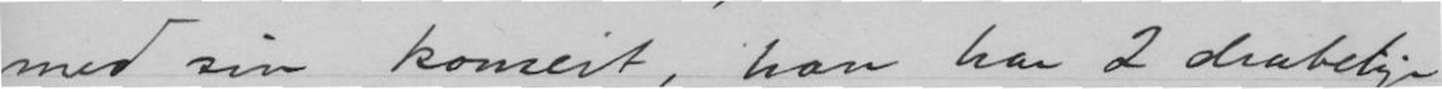med sin konsert, han har 2 drabelige
